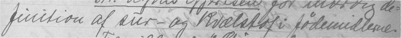finition af sur- og kvælstof i fødemidlerne.
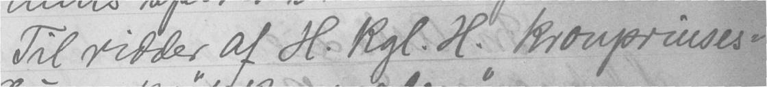Til ridder af H. Kgl. H. kronprinses-
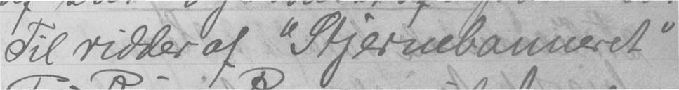Til ridder af "Stjernebanneret"
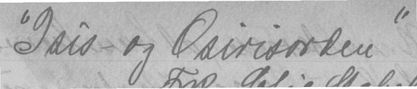"Isis - og Osirisorden"
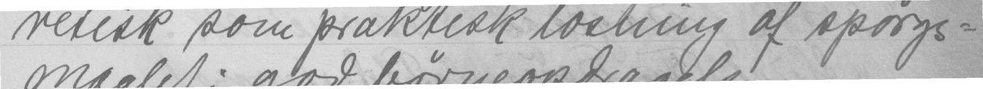retisk som praktisk løsning af spørgs-
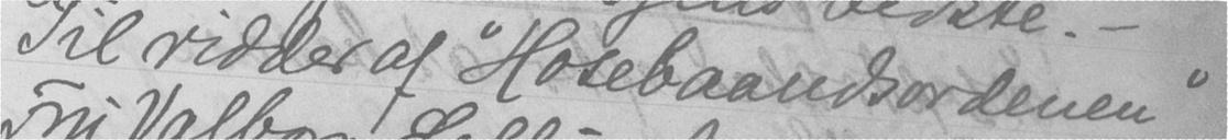Til ridder af "Hosebaandsordenen"
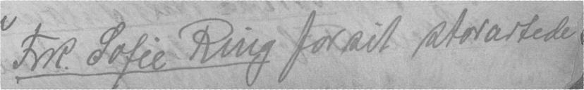Frk. Sofie Ring for sit storartede
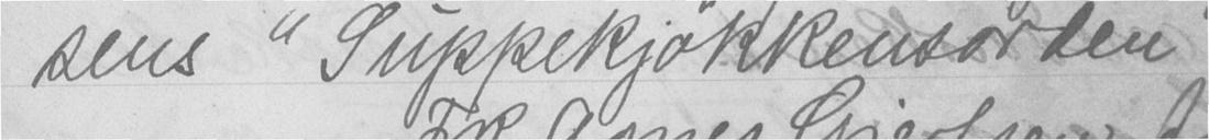sens "Suppekjøkkensorden"
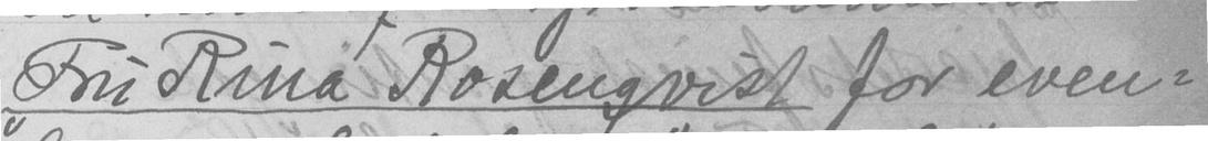Fru Rina Rosenqvist for even-
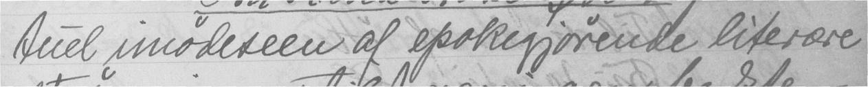tuel imødeseen af epokegjørende literære
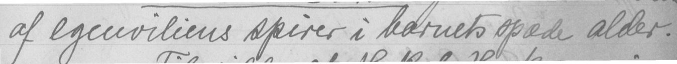af egenviliens spirer i barnets spæde alder.
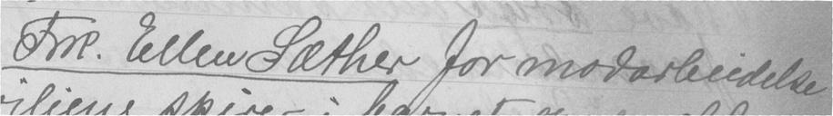Frk. Ellen Sæther for modarbeidelse
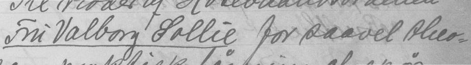Fru Valborg Sollie for saavel theo-
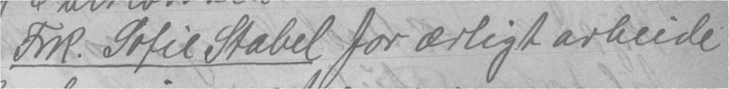Frk. Sofie Stabel for ærligt arbeide
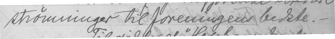strømninger til foreningens bedste. -
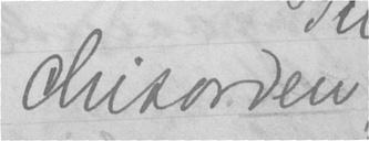chisorden
